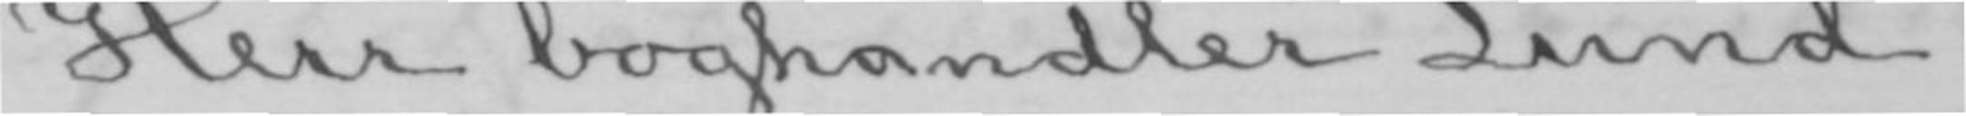Herr boghandler Lund.
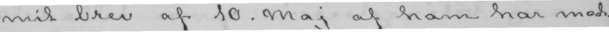mit brev af 10. Maj af ham har mod-
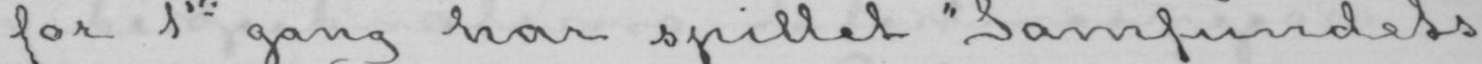for 1ste gang har spillet «Samfundets
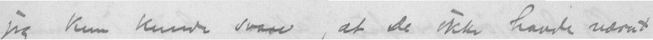jeg kun kunde svare, at De ikke havde nævnt
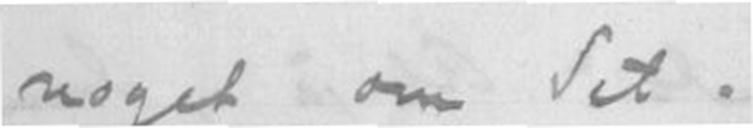noget om det.
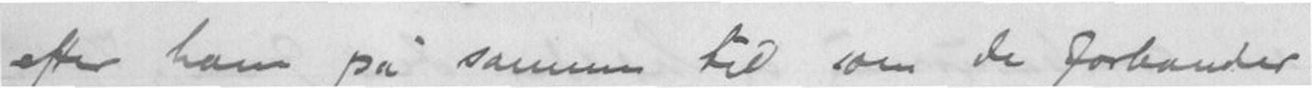efter ham på samme tid som De forbander
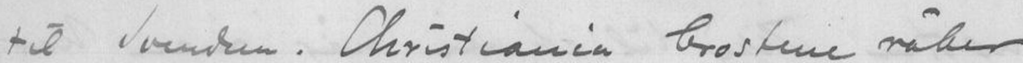til Svendsen. Christiania brostene råber
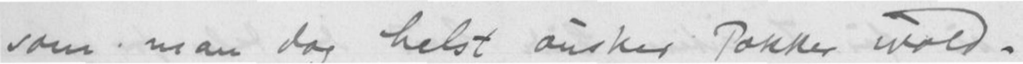som man dog helst ønsker Pokker ivold.
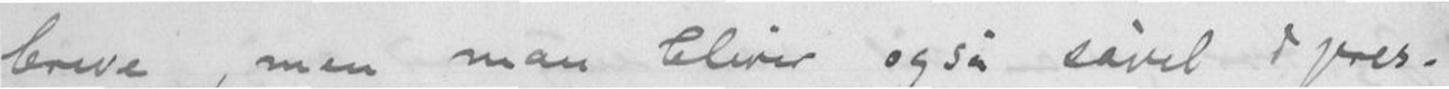breve, men man bliver også såvel i pres-
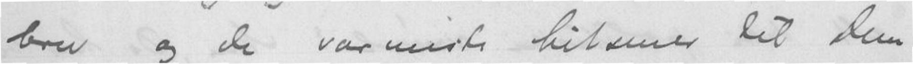brev og de varmeste hilsener til Dem
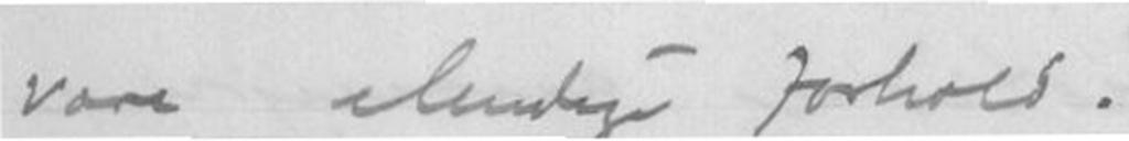vore elendige forhold.
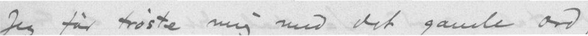Jeg får trøste mig med det gamle ord,
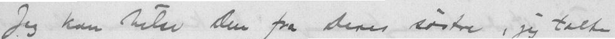Jeg kan hilse Dem fra Deres søster, jeg talte
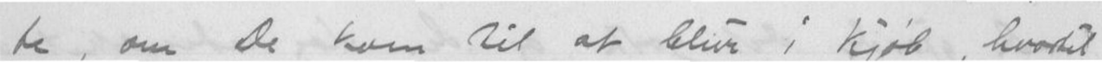te, om De kom til at blive i Kjøb, hvortil
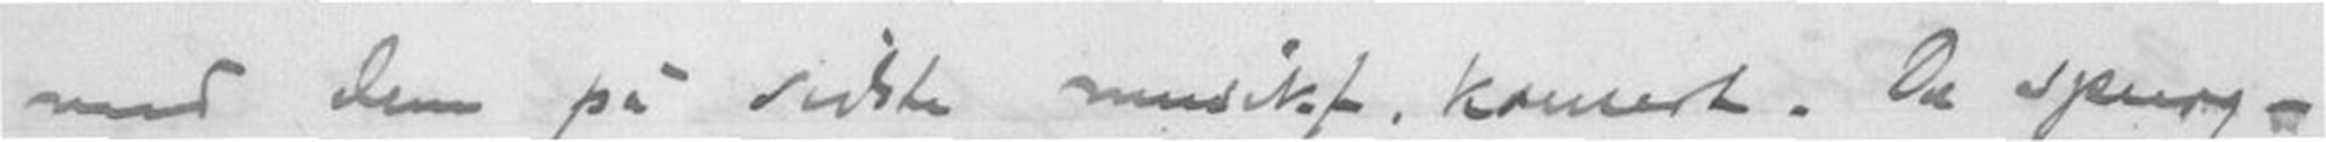med Dem på sidste musikf.konsert. De spurg-
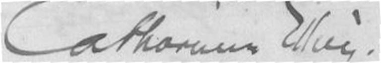Catharinus Elling.
